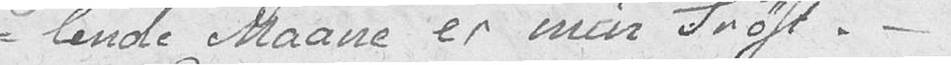-lende Maane er min Trøst. –
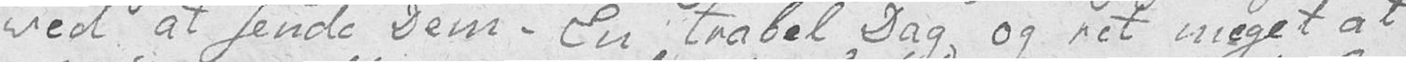ved at sende Dem. En trabel Dag, og ret meget at
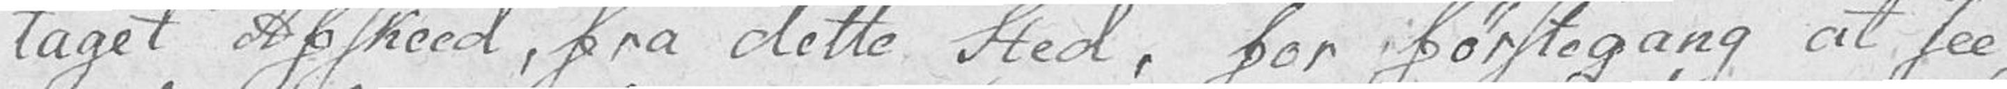taget Afskeed, fra dette Sted, for førstegang at see
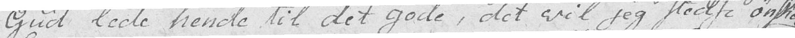Gud lede hende til det gode, det vil jeg stedse ønske.
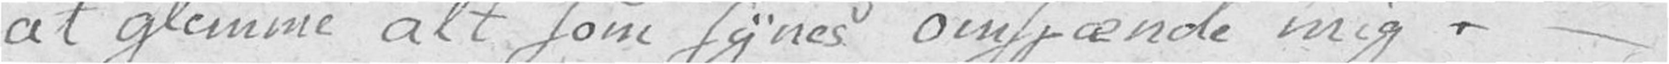at glemme alt som synes omspænde mig. –
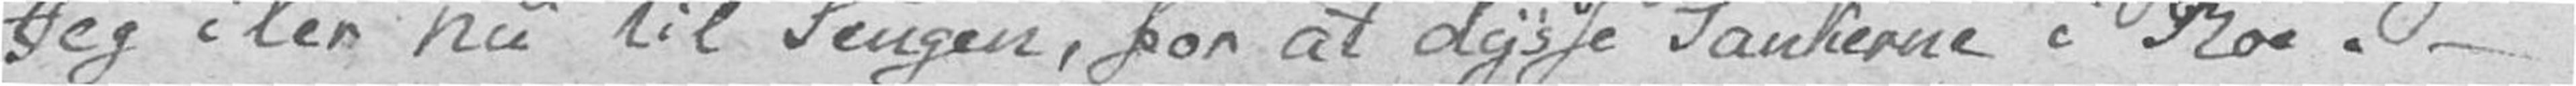Jeg iler nu til Sengen, for at dysse Tankerne i Roe. –
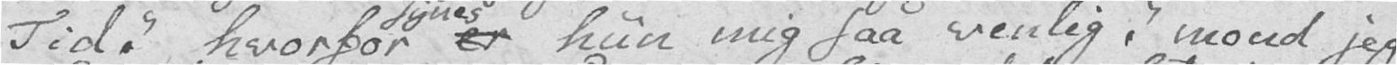Tid? hvorfor synes er hun mig saa venlig? mond jeg
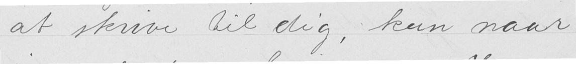at skrive til dig, kun naar
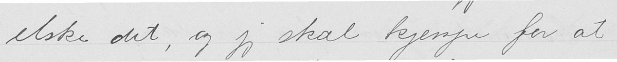elske det, og jeg skal kjempe for at
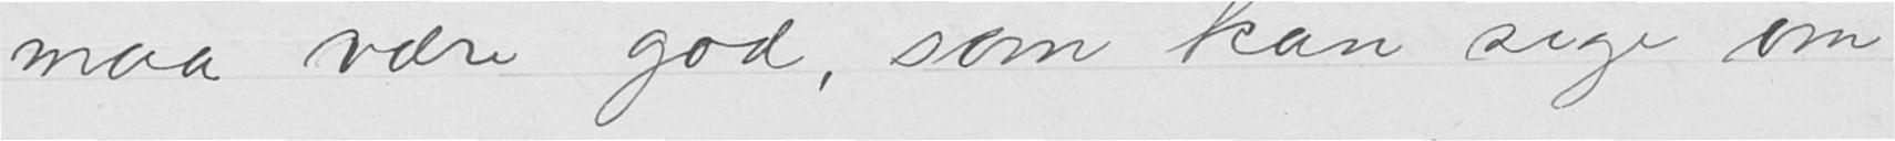maa være god, som kan sige om
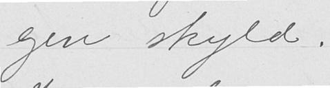egen skyld.
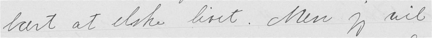lært at elske livet. Men jeg vil
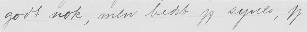godt nok, men bedst jeg synes, jeg
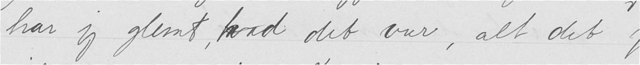har jeg glemt, hvad det var, alt det jeg
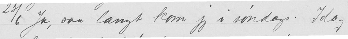25/6 Ja, saa langt kom jeg i søndags. Idag
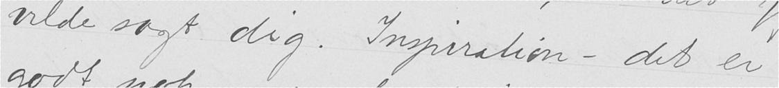vilde sagt dig. Inspiration - det er
